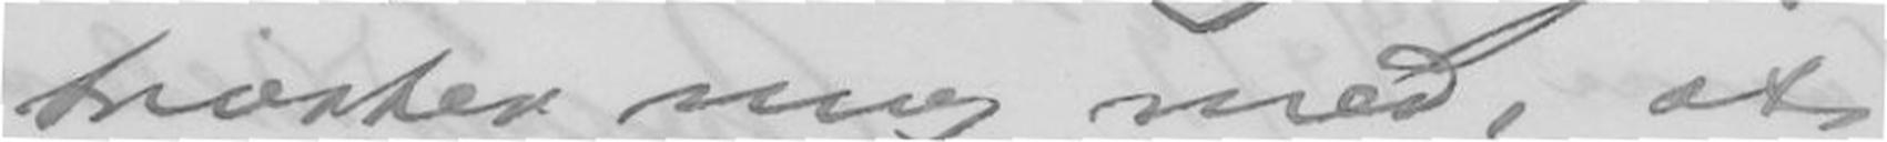Trøster mig med, at
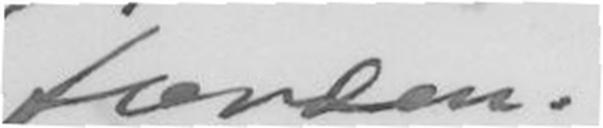færden".
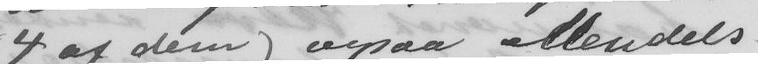(4 af dem) ogsaa Mendels-
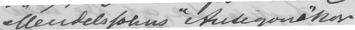Mendelsohns "Antigons"kor
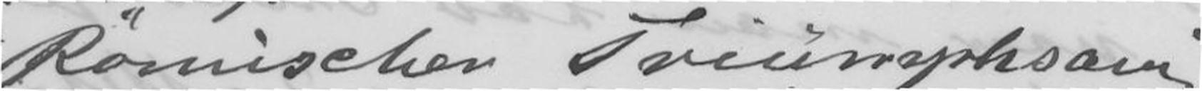"Rømischer Triumphsang".
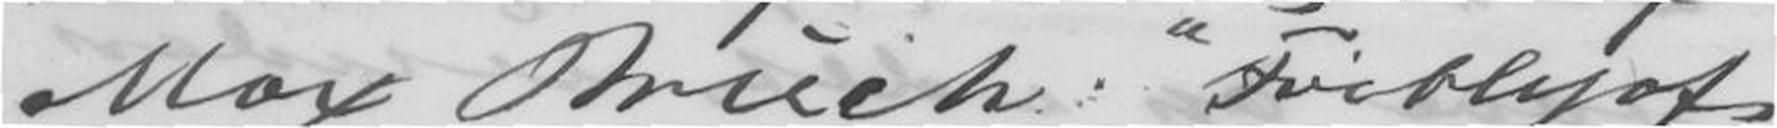Max Bruch. "Frithjofs
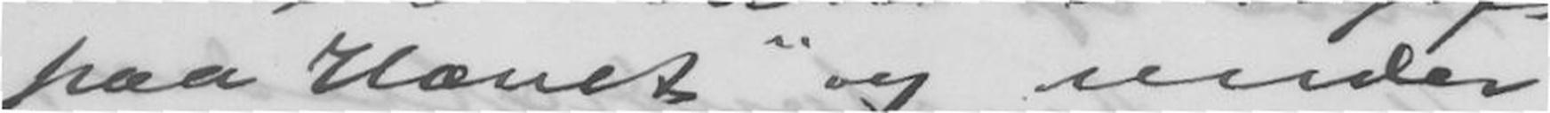paa Hænet" og under
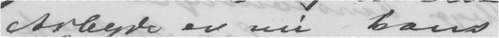Arbejde er nu hans
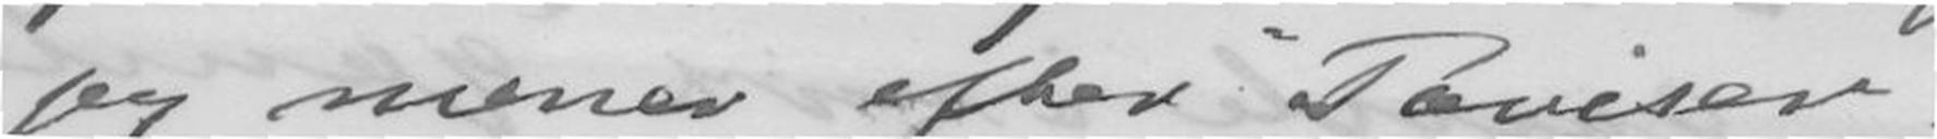jeg mener efter "Pariser
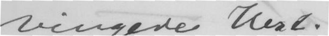vingede Hest!
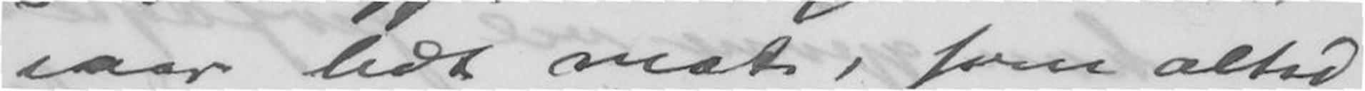iaar lidt mat, som altid
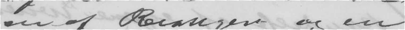er af Reissiger og en
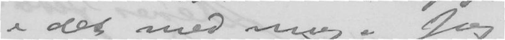i det med mig. Jeg
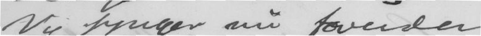Vi synger nu foruden
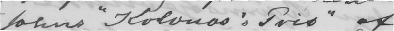sohns "Kolonos's Trio" af
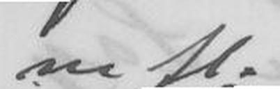m.fl.
